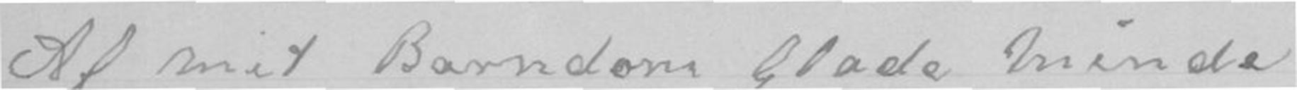Af mit Barndom Glade Minder
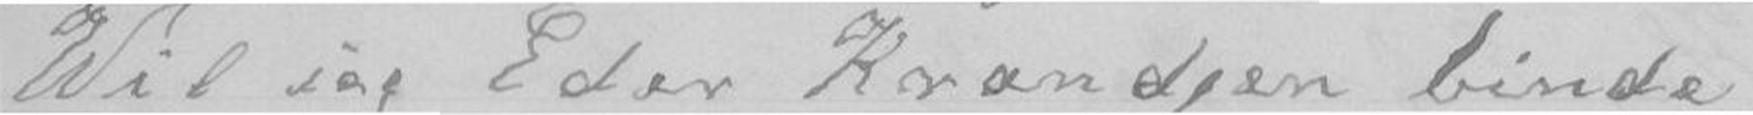Wil ieg Eder Kransdsen binde
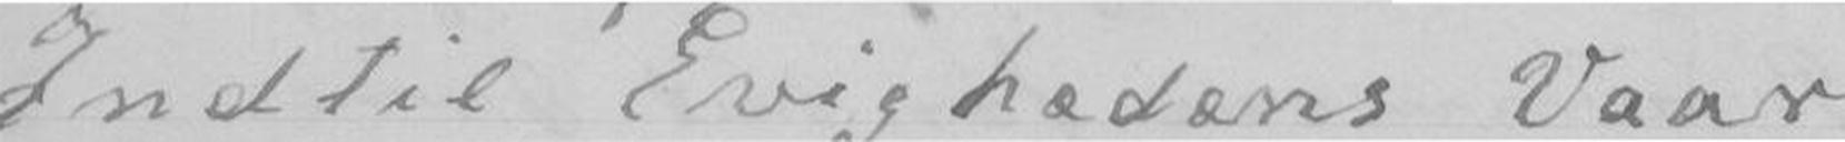Indtil Evighedens Vaar
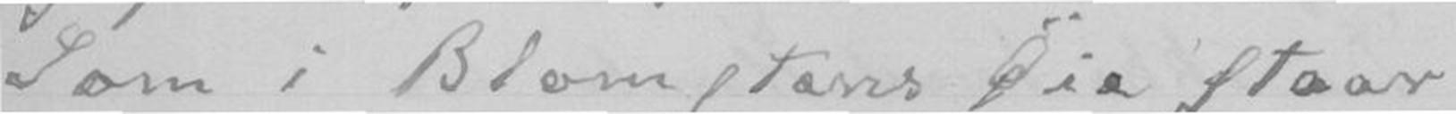Som i Blomstens Øie staar
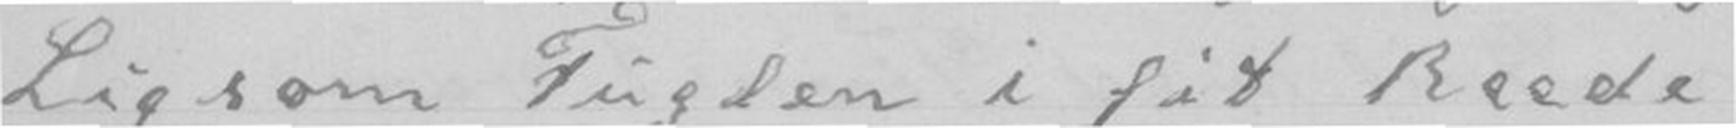Ligsom Fuglen i sit Reede
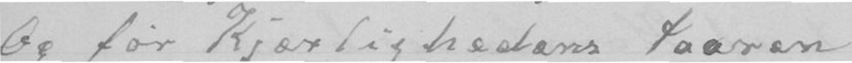Og før Kjærlighedens Saaren
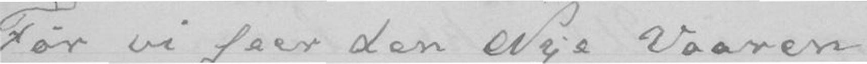Før vi seer den Nye Vaaren
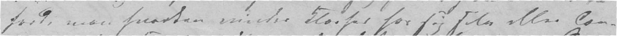fordi man hverken vinder Klarhed for sig selv eller Con-
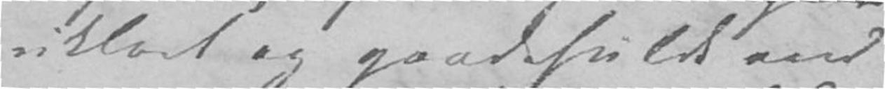uklart og gaadefuldt end
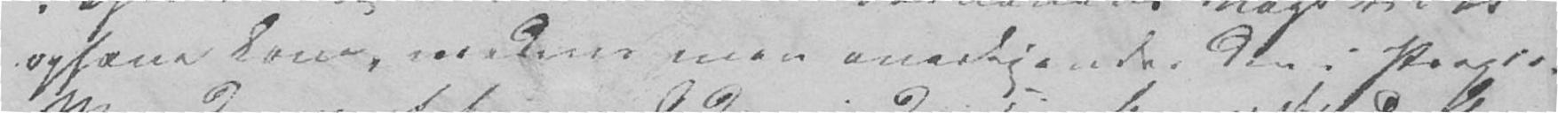ophæve Love, medens man anerkjender den i Praxis.
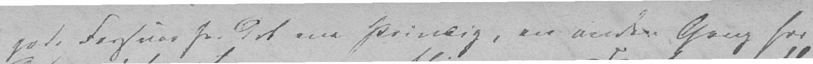godt Forsvar for det ene Princip, en anden Gang for
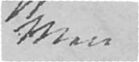Men
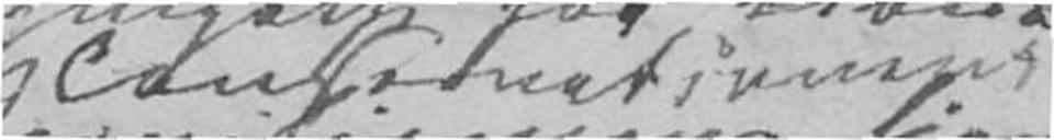Conservatismens
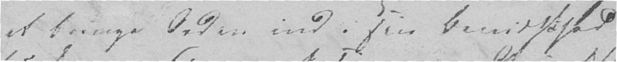at bringe Orden ind i sin Bevidsthed
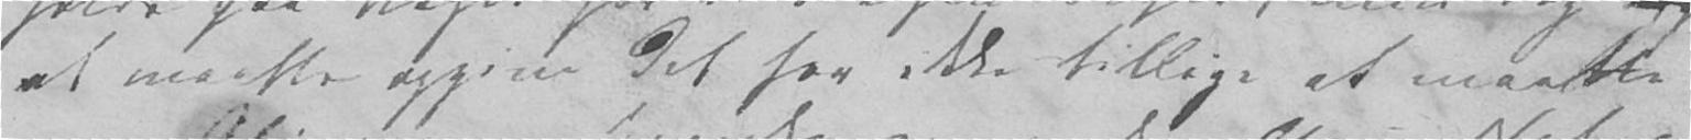at maatte opgive det for ikke tillige at maatte
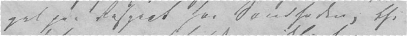gel paa Respect for Sandheden; thi
